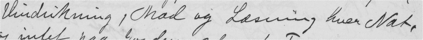Vindrikning, Mad og Læsning hver Nat,
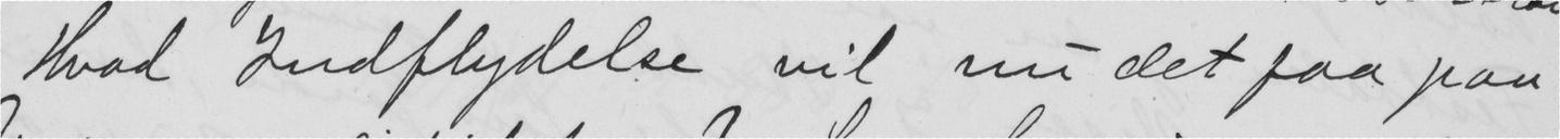Hvad Indflydelse vil nu det faa paa
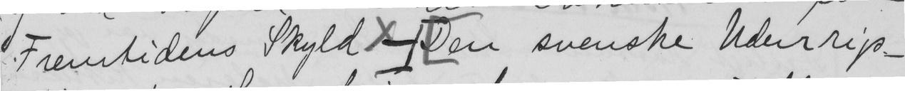Fremtidens Skyld  Den svenske Uderrigs-
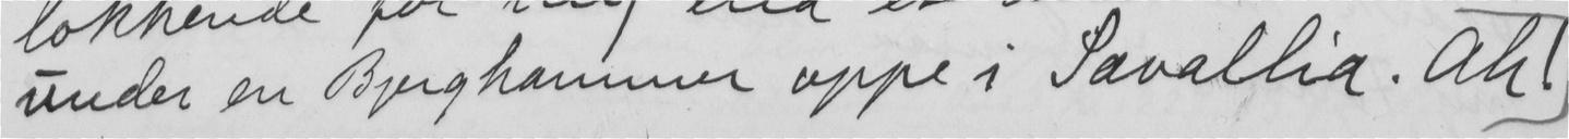under en Bjerghammer oppe i Savallia. Ak!
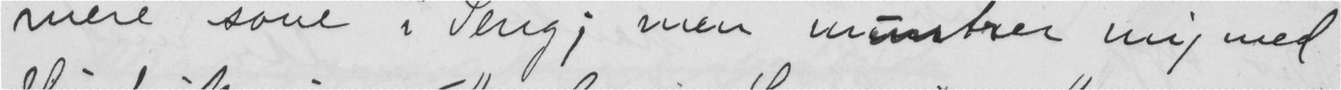mere sove i Seng; men muntrer mig med
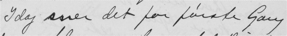Idag sner det for første Gang
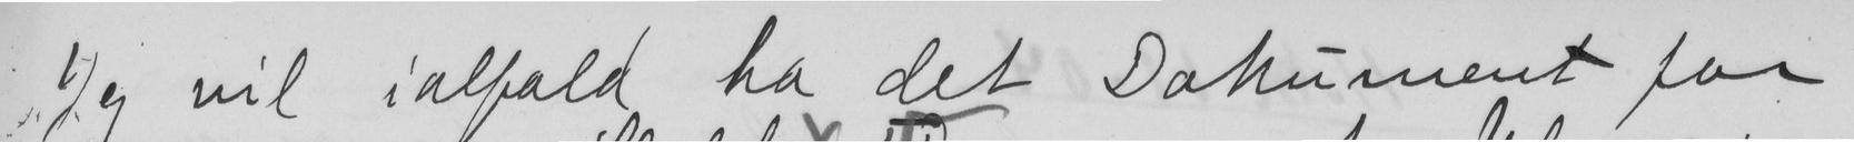Jeg vil ialfald ha det Dokument for
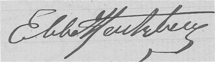Ebbe Hertzberg
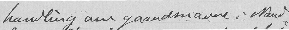handling om gaardsnavne i Nord-
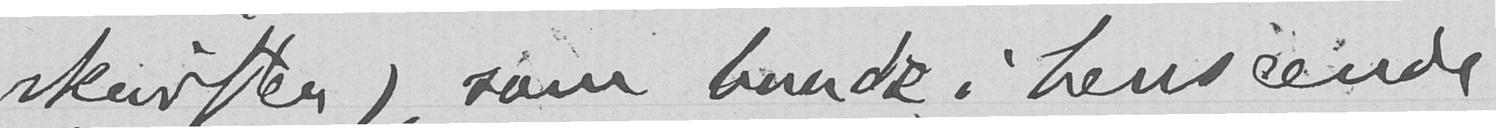skrifter), som baade i henseende
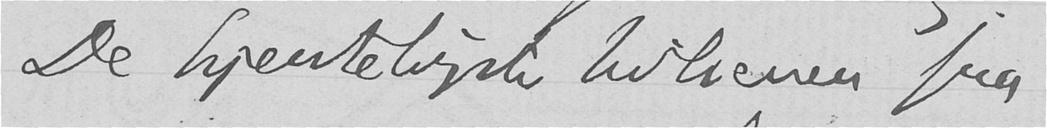De hjerteligste hilsener fra
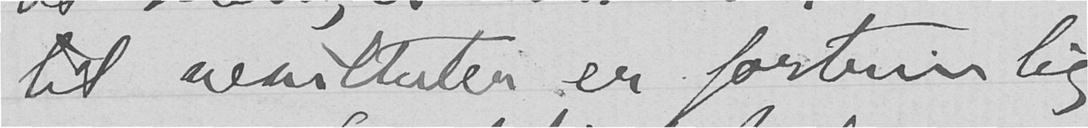til resultater er fortrinlig.
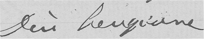Din hengivne
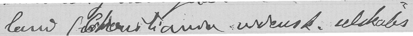land (Christiania vidensk. selskabs
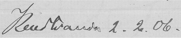Rendtranda 2. 2. 06.
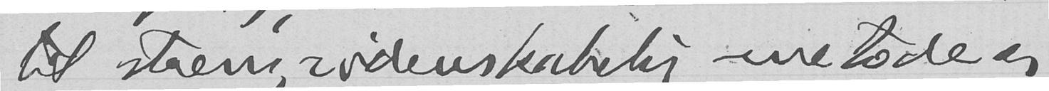til streng, videnskabelig metode og
